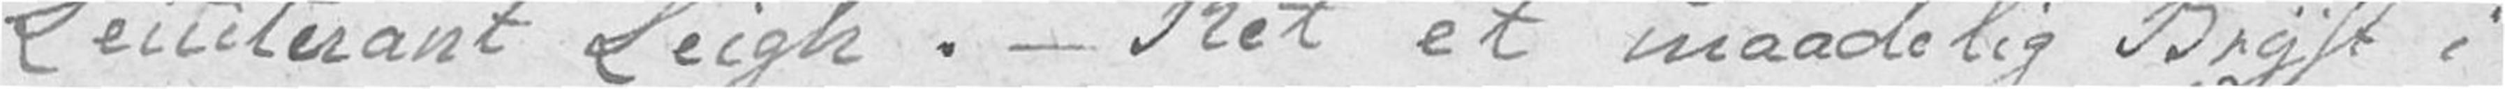Leiutnant Leigh. – Ret et maadelig Bryst i
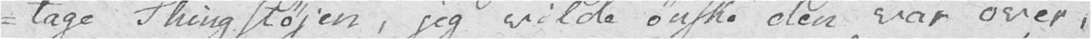-tage Thingstøjen, jeg vilde ønske den var over,
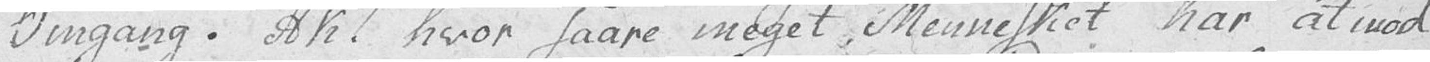Omgang. Ak! hvor saare meget Mennesket har at mod
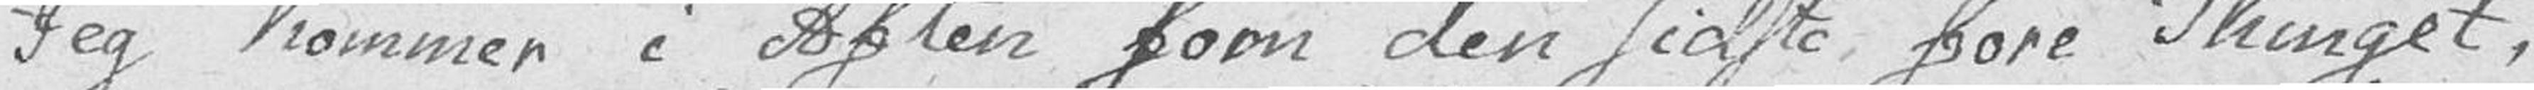Jeg kommer i Aften som den sidste fore Thinget,
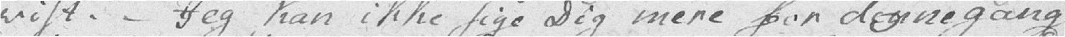vist. – Jeg kan ikke sige Dig mere for dennegang
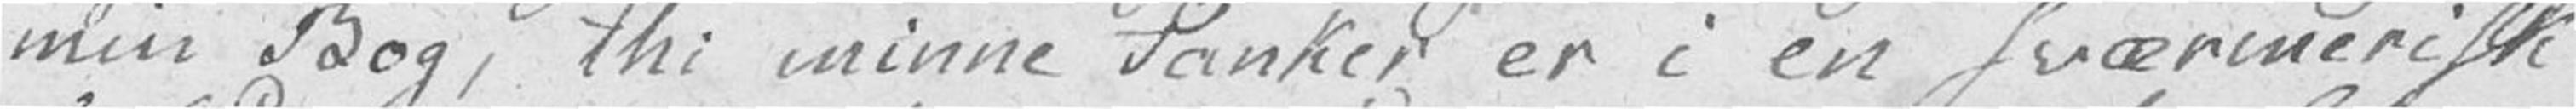min Bog, thi minne Tanker er i en sværmerisk
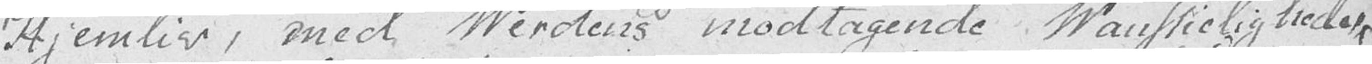Hjemliv, med Verdens modtagende Vanskeligheder,
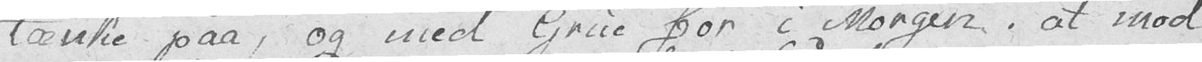tænke paa, og med Grue for i Morgen, at mod
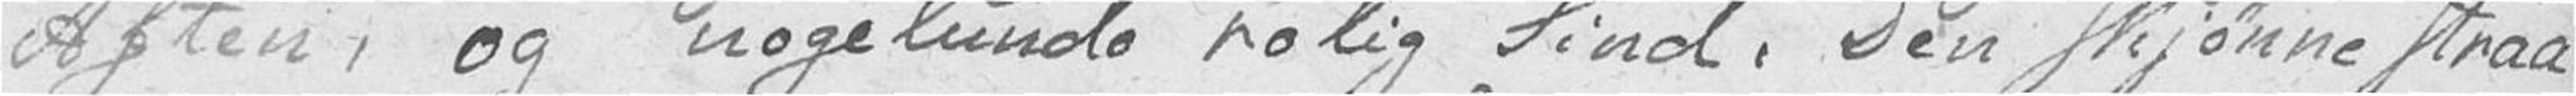Aften, og nogenlunde rolig Sind. Den skjønne straa
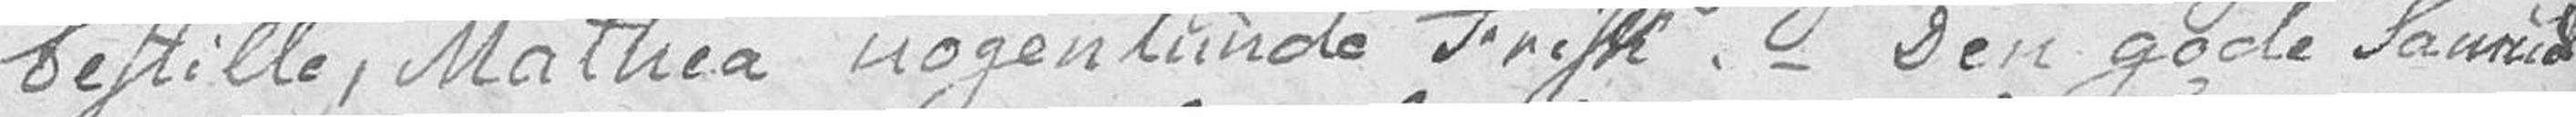bestille, Mathea nogenlunde Frisk. – Den gode Samuel
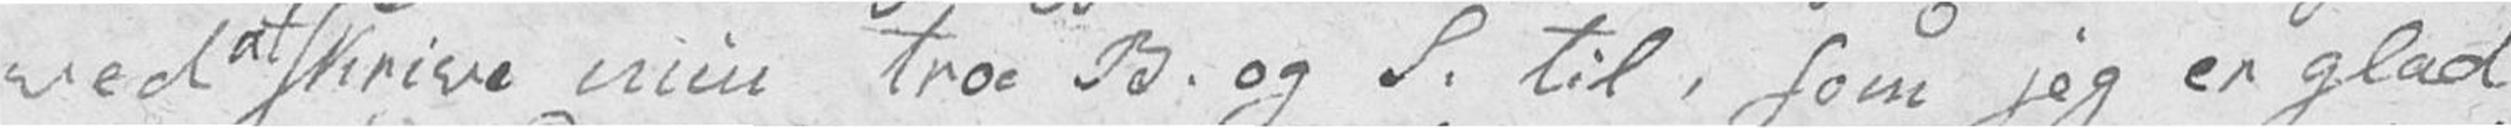ved at skrive min troe B. og S. til, som jeg er glad
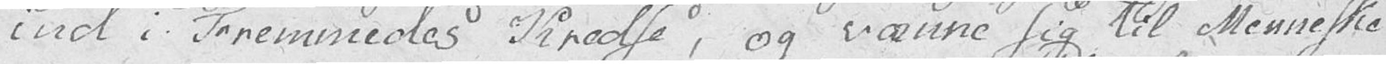ind i Fremmedes Kredse, og vænne sig til Menneske
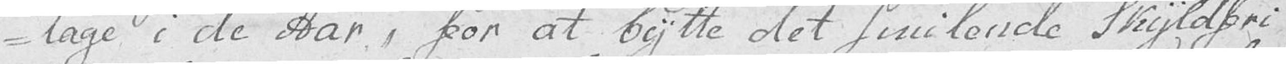-tage i de Aar, for at bytte det smilende Skyldfri
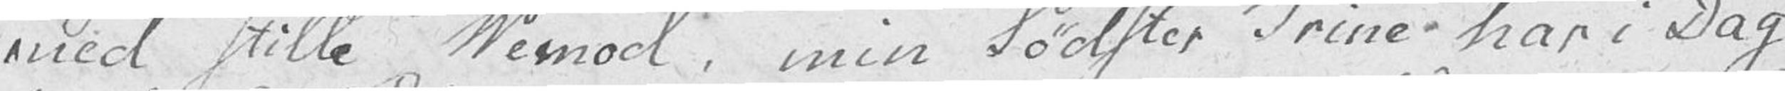med stille Vemod, min Sødster Trine har i Dag
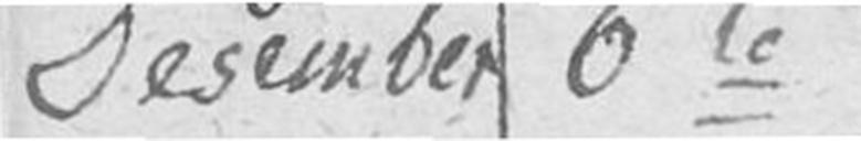Desember 6te
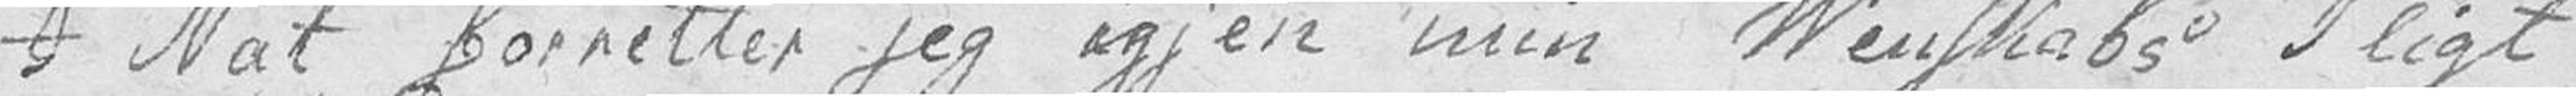I Nat forretter jeg igjen min Venskabs Pligt
